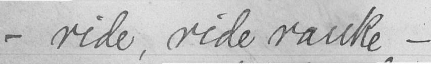- ride, ride ranke -
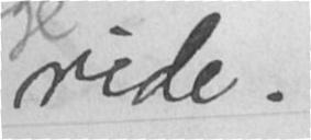ride.
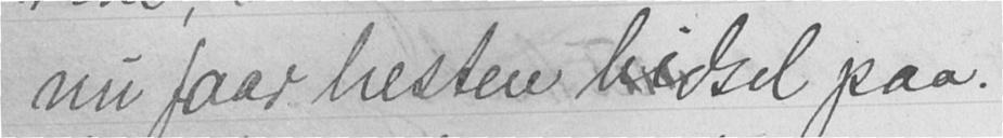nu faar hesten bidsel paa.
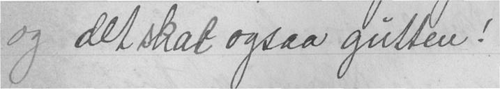og det skal ogsaa gutten!
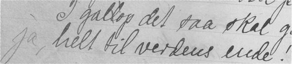ja, helt til verdens ende!
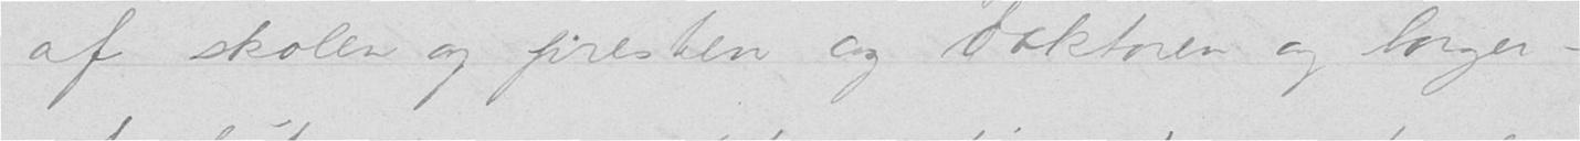af skolen og presten og doktoren og borger-
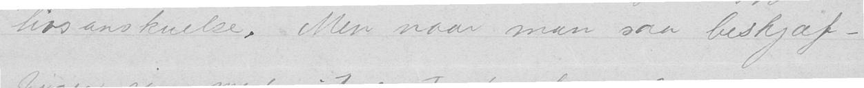livsanskuelse. Men naar man saa beskjæf-
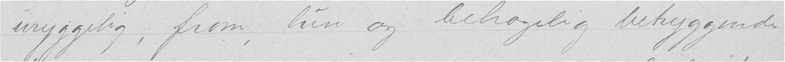uhyggelig, from, lun og behagelig betryggende
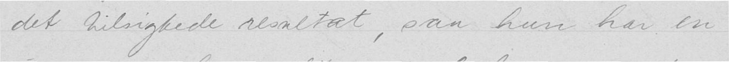det tilsigtede resultat, saa hun har en
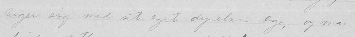tiger sig med sit eget dyrebare ego, og man
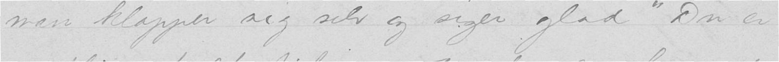man klapper sig selv og siger glad "Du er
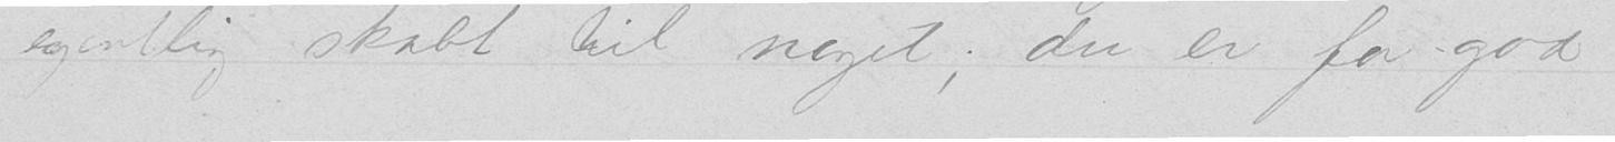egentlig skabt til noget, du er for god
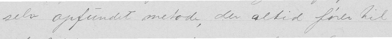selv opfundet metode, der altid fører til
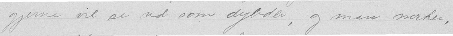gjerne vil se ud som dybder, og man merker,
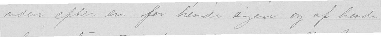uden efter en for hende egen og af hende
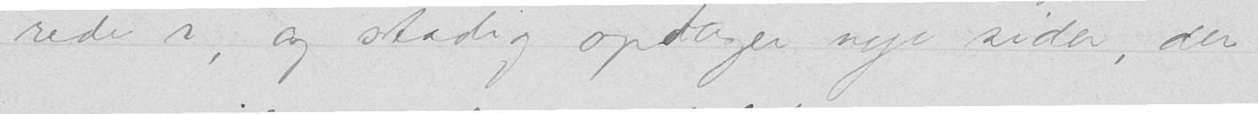rede i, og stadig opdager nye sider, der
